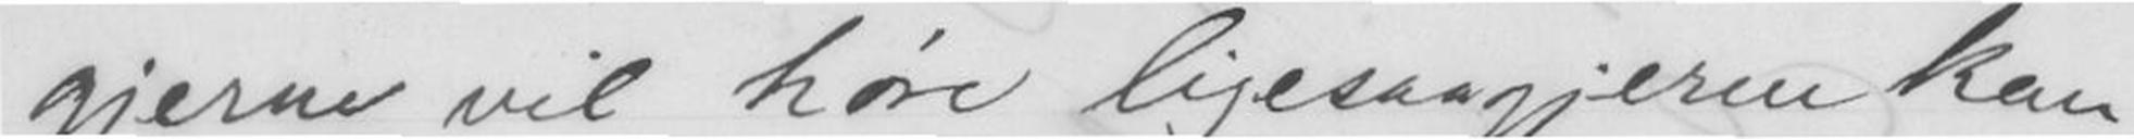gjerne vil høre ligesaagjerne kan
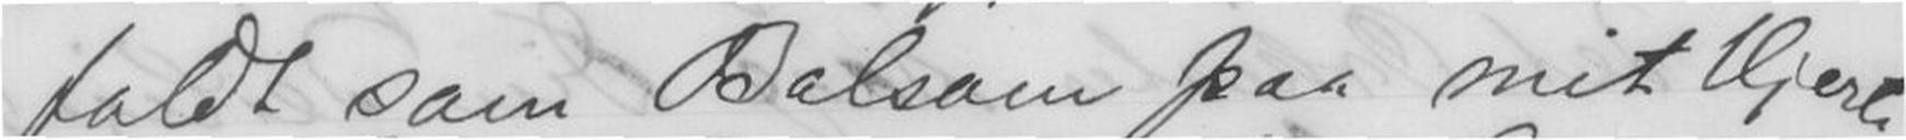faldt som Balsam paa mit Hjerte
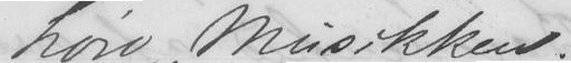høre Musikken.
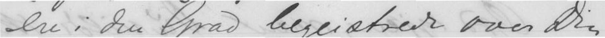ere i den Grad begeistrede over Din
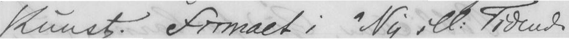Kunst. Firmaet; "Ny ill Tidende
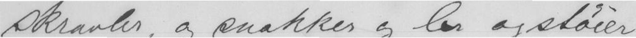skravler, og snakker og ler og støier
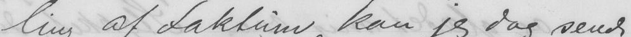ling af Faktum, kan jeg dog sende
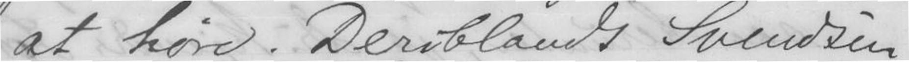at høre. Deriblandt Svendsen
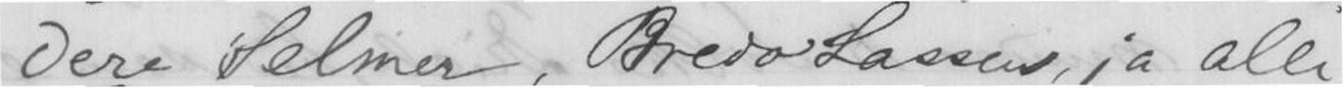videre Selmer, Bredo Lassen, ja alle
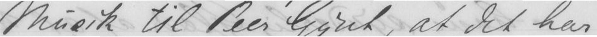Musik til Peer Gynt, at det har
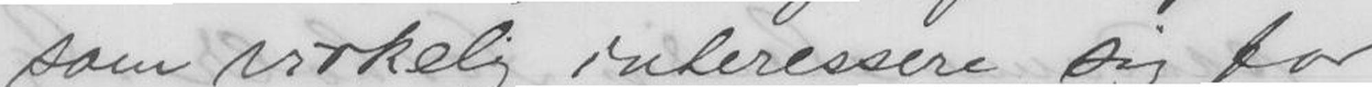som virkelig interessere sig for
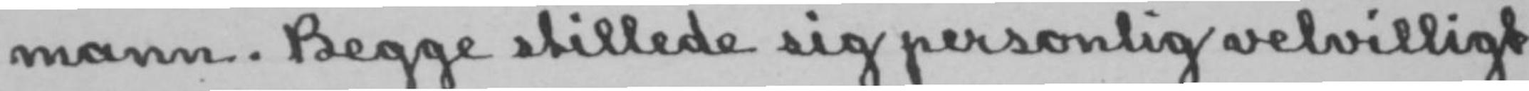mann. Begge stillede sig personlig velvilligt
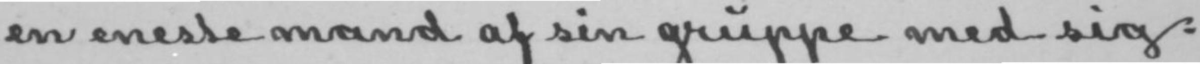en eneste mand af sin gruppe med sig.
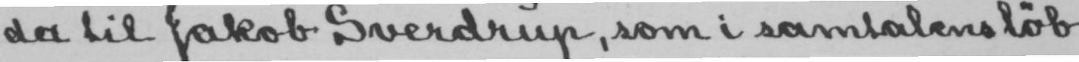dea til Jakob Sverdrup, som i samtalens løb
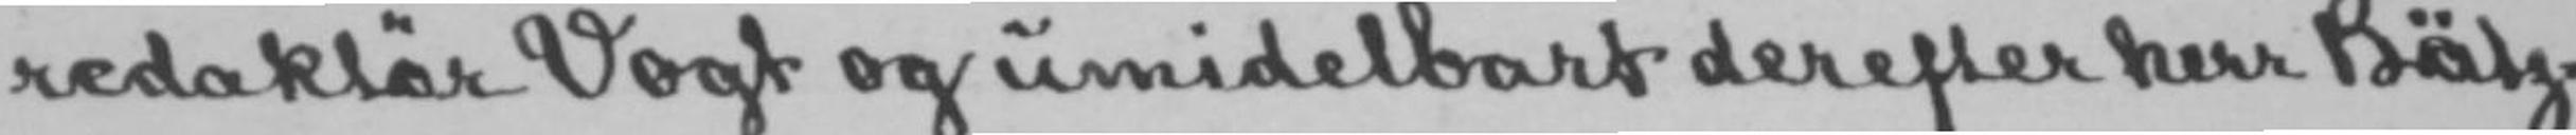redaktør Vogt og umidelbart derefter herr Bätz-
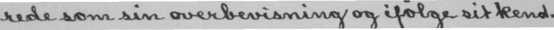rede som sin overbevisning og ifølge sit kend-
